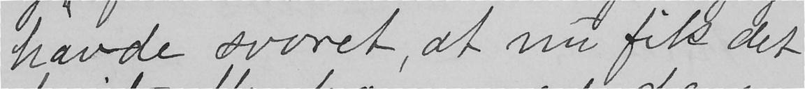havde svoret, at nu fik det
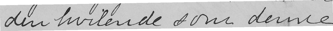den hvilende som denne
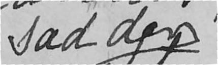sad der
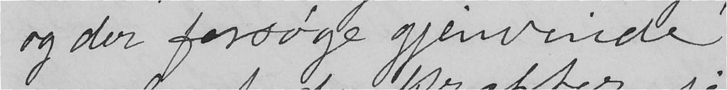og der forsøge gjenvinde
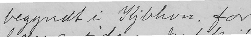begyndt i Kjbhvn. for
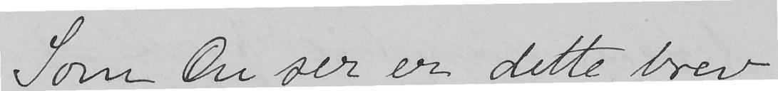Som Du ser er dette brev
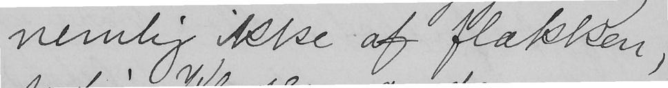nemlig ikke af flækken,
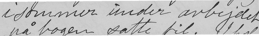i sommer under arbejdet
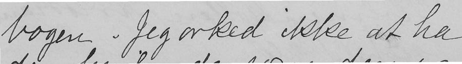bogen. Jeg orked ikke at ha
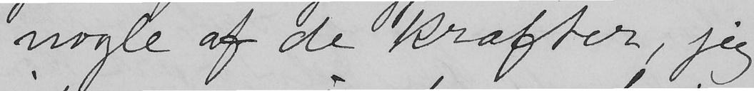nogle af de kræfter, jeg
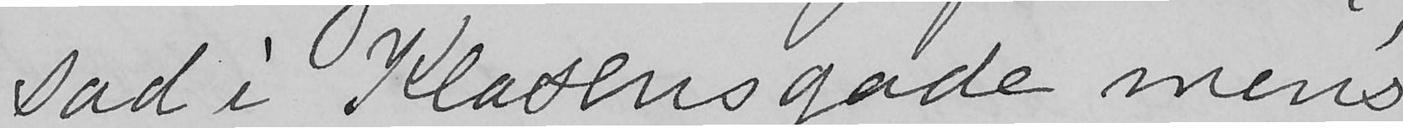sad i Klasensgade mens
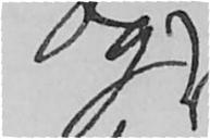og
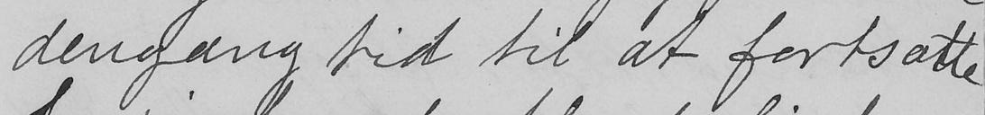dengang tid til at fortsætte
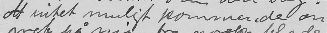At intet muligt kommende an
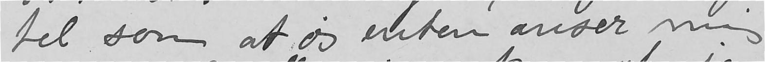tel som at os inten anser mig
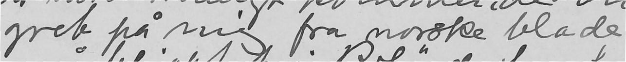greb på mig fra norske blade
